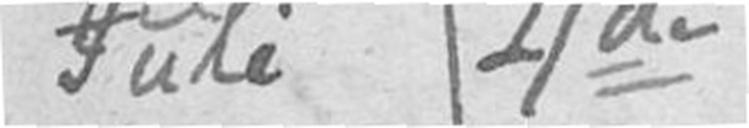Juli 4de
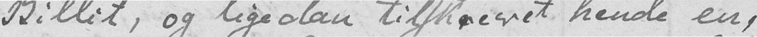Billit, og ligedan tilskrevet hende en,
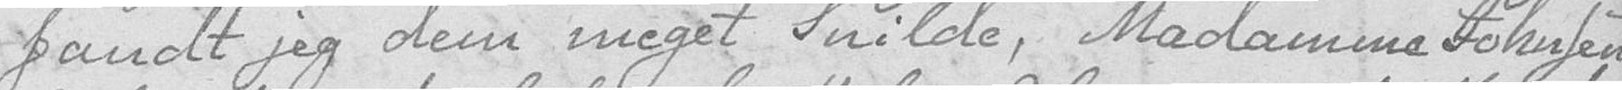fandt jeg dem meget Snilde, Madamme Johnsen
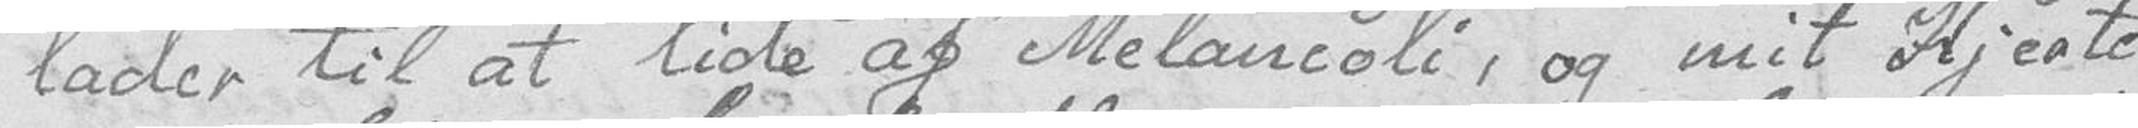lader til at lide af Melancoli, og mit Hjerte
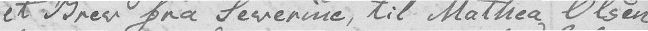et Brev fra Severine, til Mathea Olsen
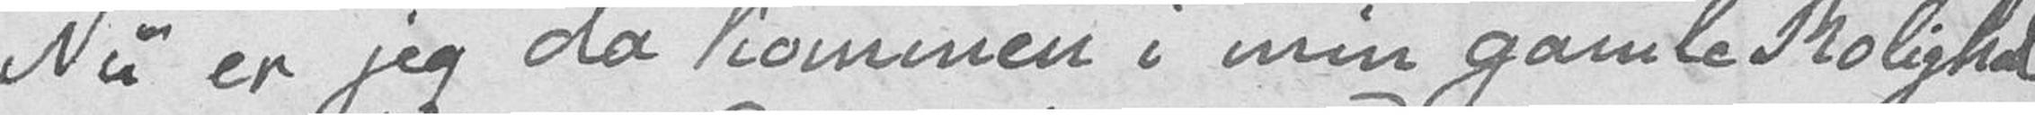Nu er jeg da kommen i min gamle Rolighed
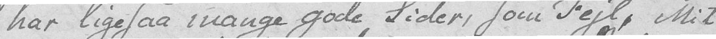har ligesaa mange gode Sider, som Fejl, Mit
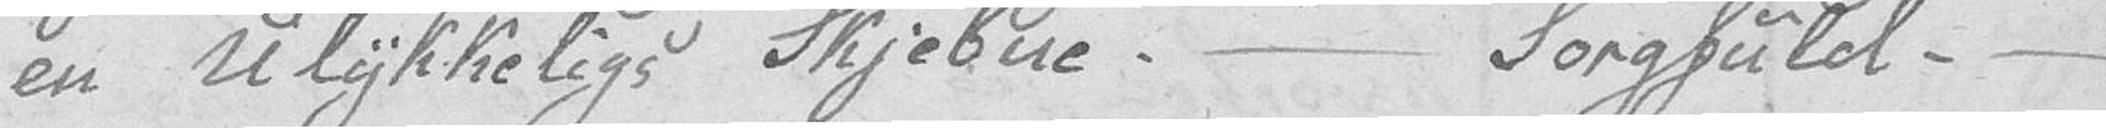en ulykkeligs Skjebne. – Sorgfuld. –
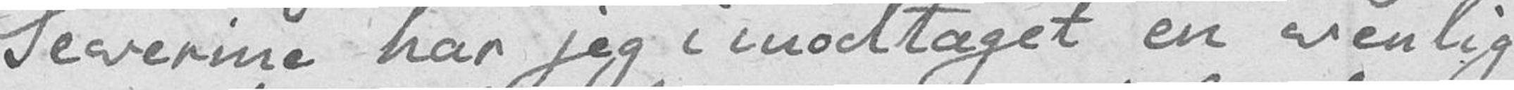Severine har jeg imodtaget en venlig
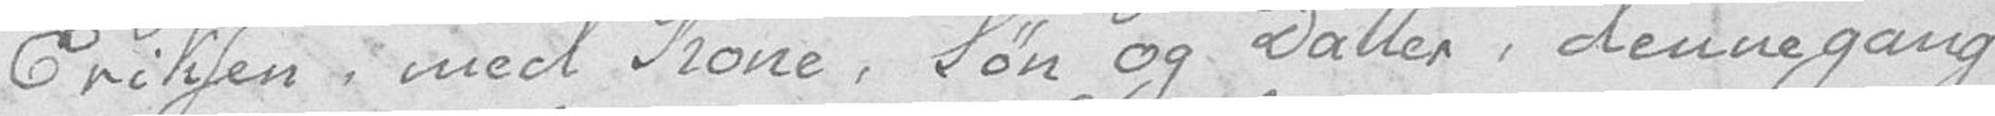Eriksen, med Kone, Søn og Datter, dennegang
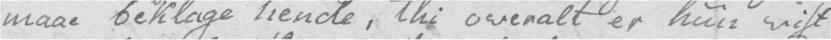maae beklage hende, thi overalt er hun vist
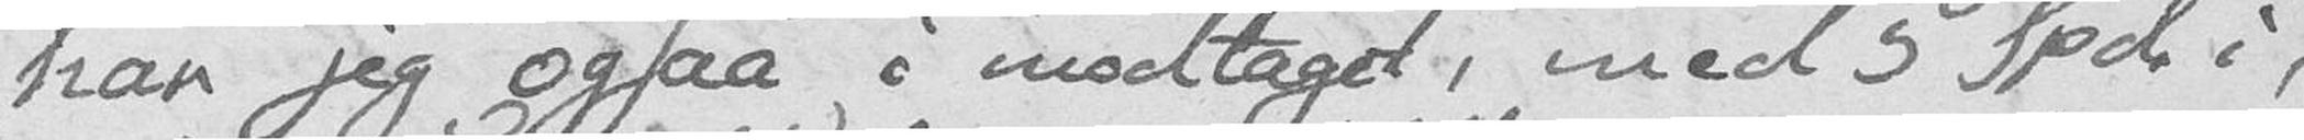har jeg ogsaa i modtaget, med 5 Spd. i,
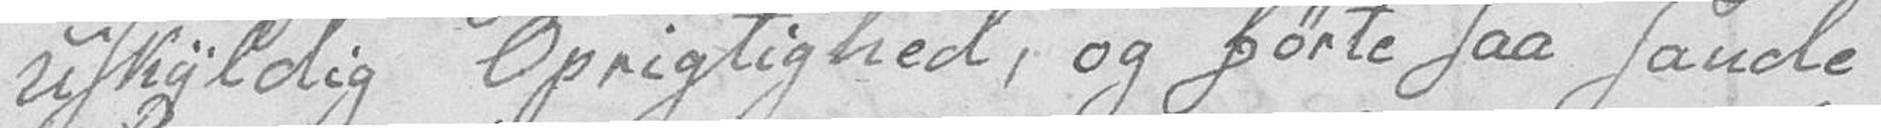uskyldig Oprigtighed, og førte saa sande
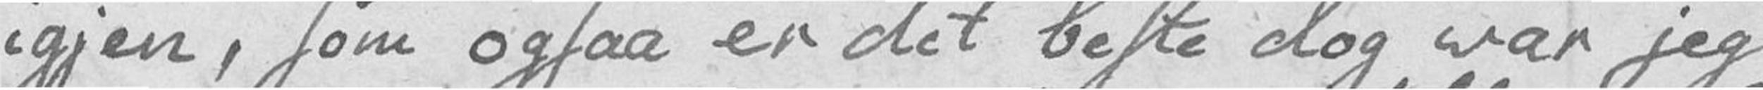igjen, som ogsaa er det beste dog var jeg
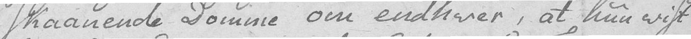skaanende Domme om endhver, at hun vist
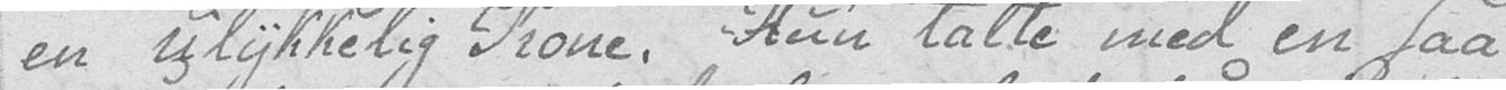en ulykkelig Kone. Hun talte med en saa
